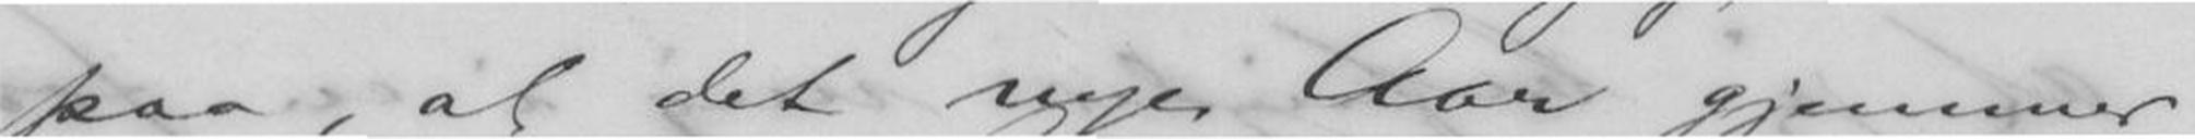paa, at det nye Aar gjemmer
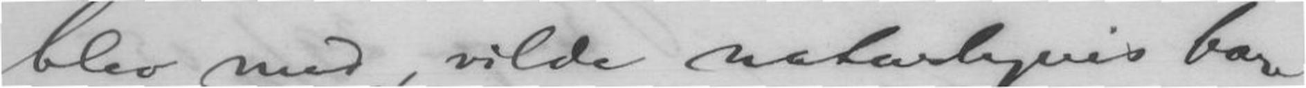blev med, vilde naturligvis bare
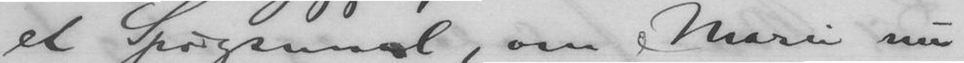et Spørgsmaal, om Marie nu
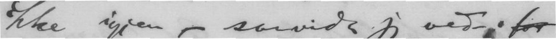ikke igjen, saavidt jeg ved-.for
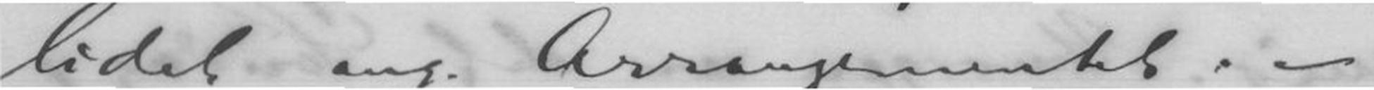lidet ang. Arrangementet. -
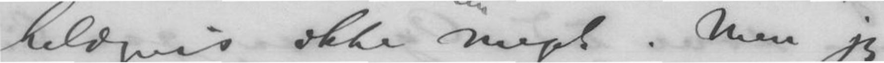heldigvis ikke meget. Men jeg
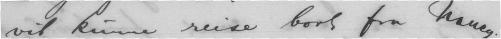vil kunne reise bort fra Nancy.
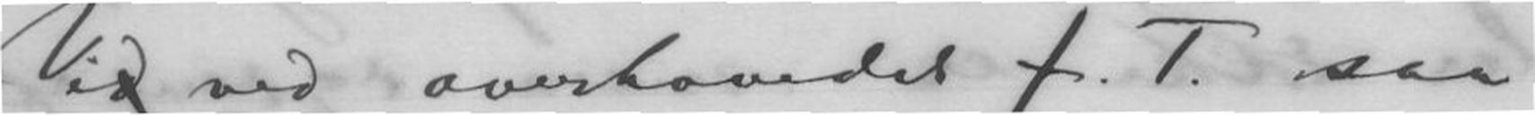Vid ved overhovedet f. T. saa
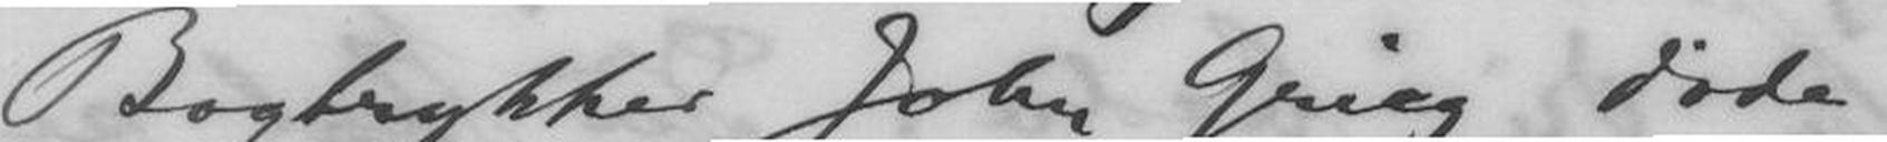Bogtrykker John Grieg døde
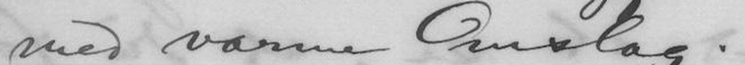med varme Omslag.
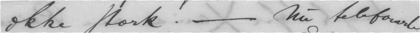ikke stærk. - Nu telefonerte
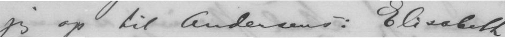jeg op til Andersens: Elisabeth
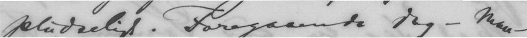pludseligt. Foregaaende dag - Man-
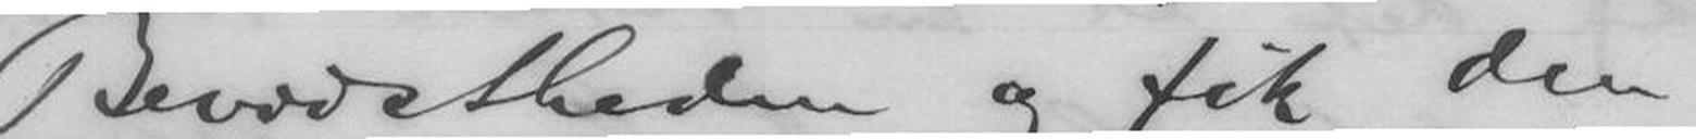Bevidstheden og fik den
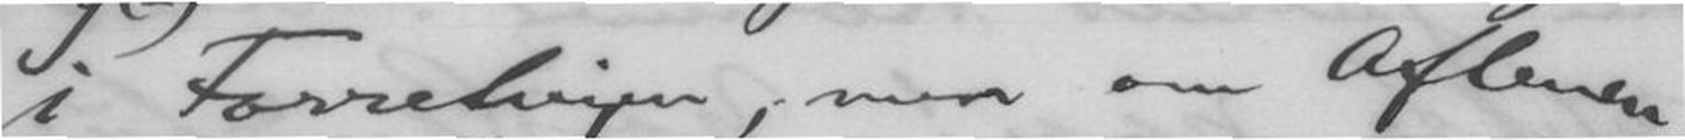i Forretningen, men om Aftenen
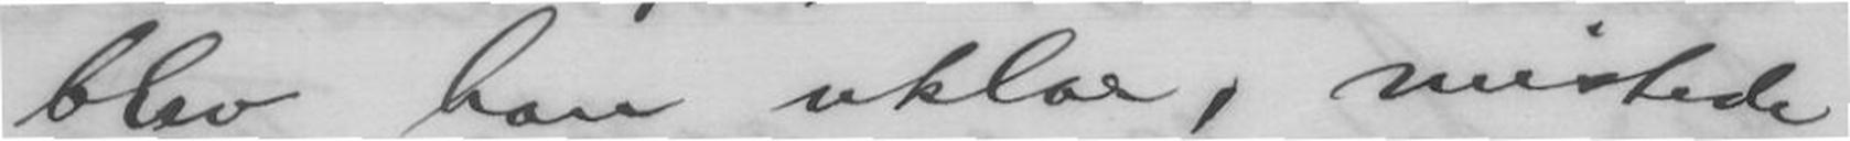blev han uklar, mistede
